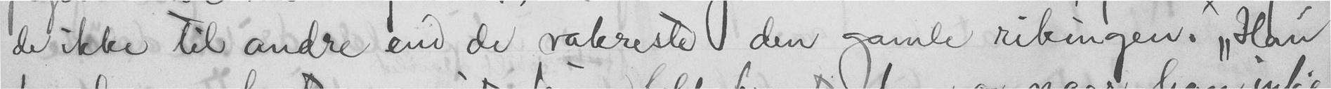de ikke til andre end de vakreste den gamle rikingen. "Han
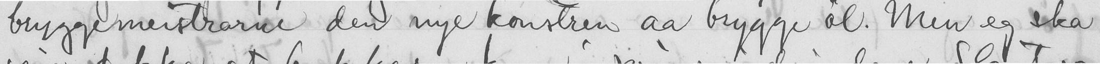bryggemeistrarne den nye konstren aa brygge øl. Men eg ska
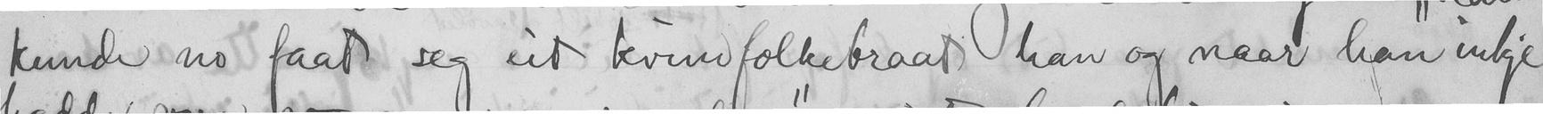kunde no faat sig eit kvindfolkebraat han og naar han inkje
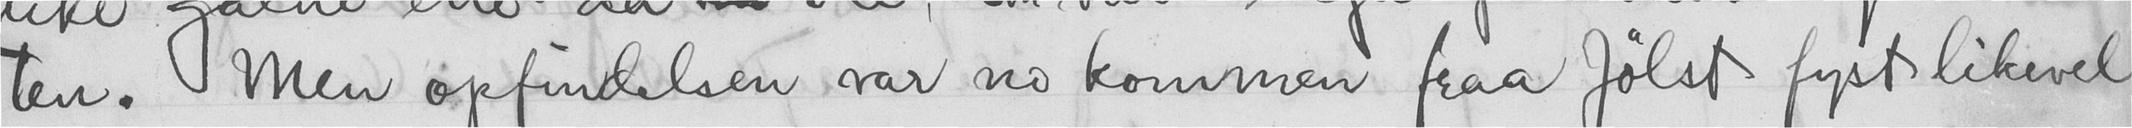ten. Men opfindelsen, var no kommen paa Jølst fyst likevel
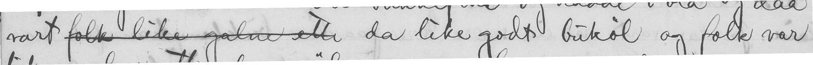vart  da like godt bukøl og folk var
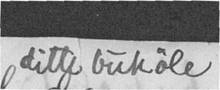ditte bukøle
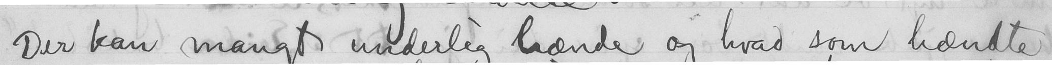Der kan mangt underlig hænde og hvad som hændte
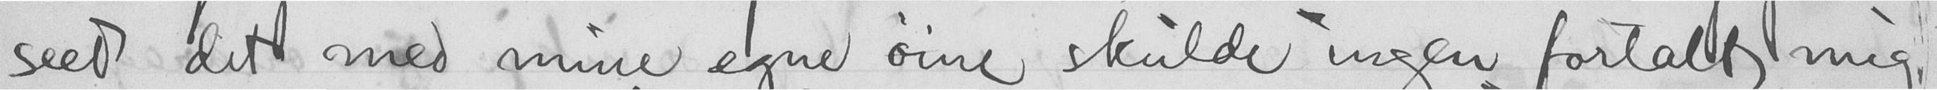seed det med mine egne øine skulde ingen fortalt mig
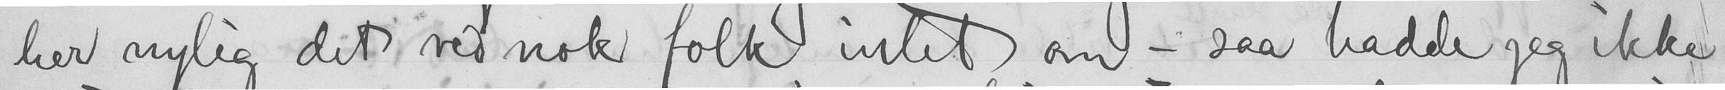her nylig det ved nok folk intet om - saa hadde jeg ikke
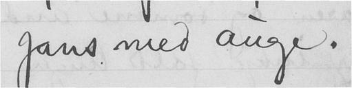Jans med auge.
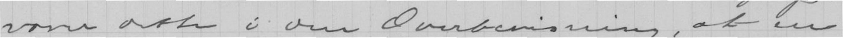vover dette i den Overbevisning, at en
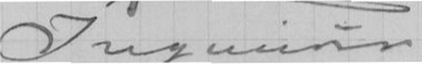Ingeniør
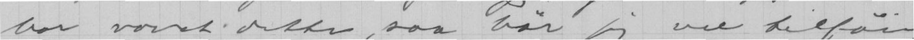har vovet dette, saa bør jeg vel tilføie,
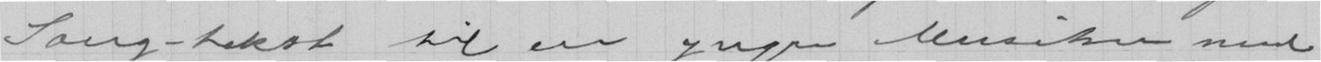Sang-tekst til en Yngre Musiker med
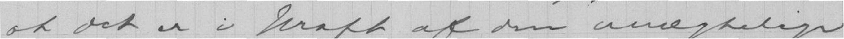at det er i kraft af den unægtelige
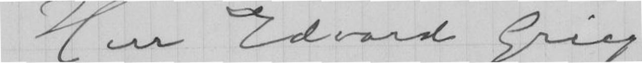Herr Edvard Grieg
